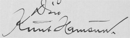Knut Hamsun.
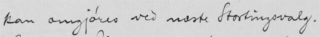kan omgjøres ved næste Stortingsvalg.
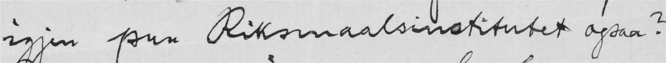igjen paa Riksmaalsinstitutet ogsaa?
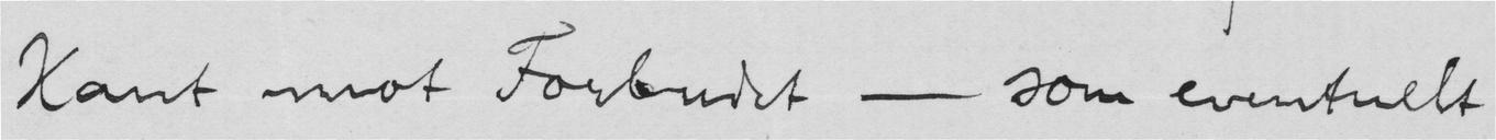Kant mot Fobudet - som eventuelt
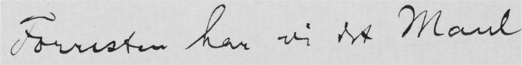Forresten har vi det Maul
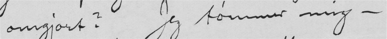omgjort? Jeg tømmer mig -
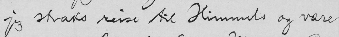jeg straks reise til Himmels og være
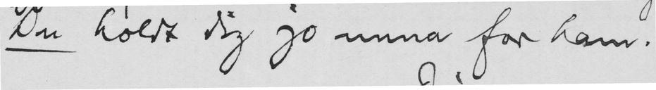Du holdt dig jo unna for ham.
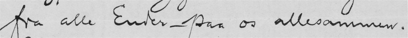fra alle Ender - paa os allesammen.
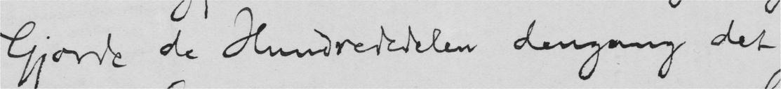Gjorde de Hundrededelen dengang det
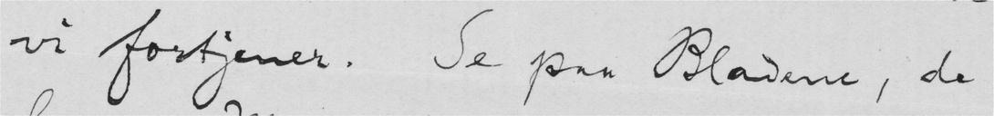vi fortjener. Se paa Bladene, de
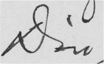Din
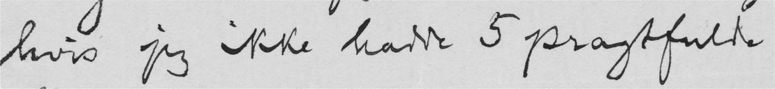hvis jeg ikke hadde 5 pragtfulde
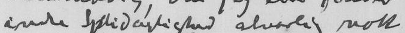indre Splidagtighed alvorlig nok.
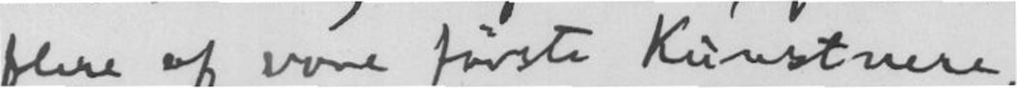flere af vore første Kunstnere,
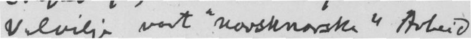Velvilje vort "norsknorske" Arbeid
